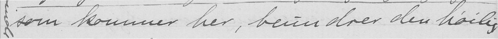som kommer her, beundrer den høilig
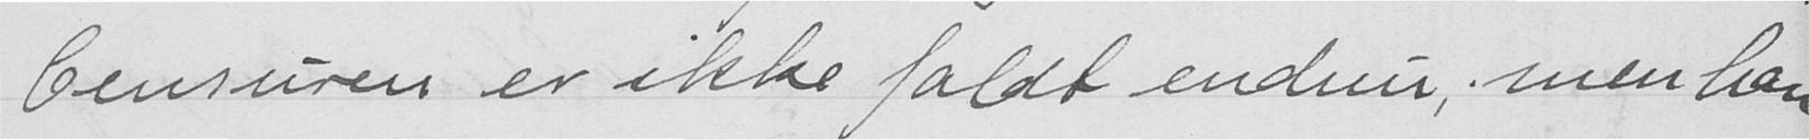Censuren er ikke faldt endnu, men han
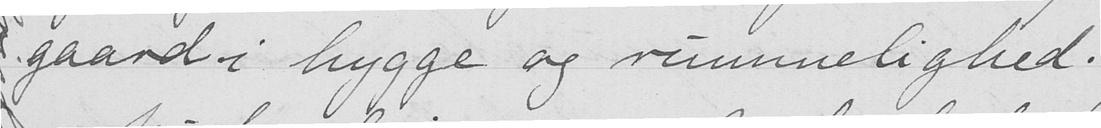gaard i hygge og rummelighed.
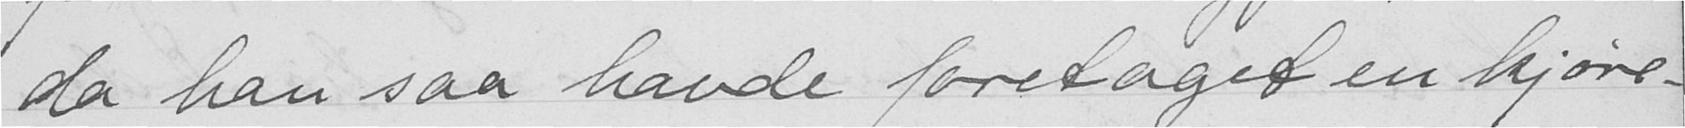da han saa havde foretaget en kjøre-
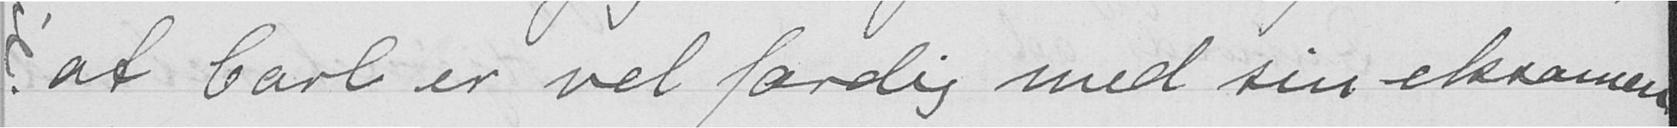at Carl er vel færdig med sin eksamen.
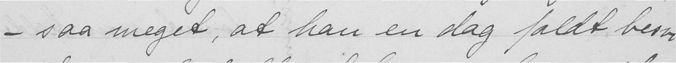- saa meget, at han en dag faldt besvi-
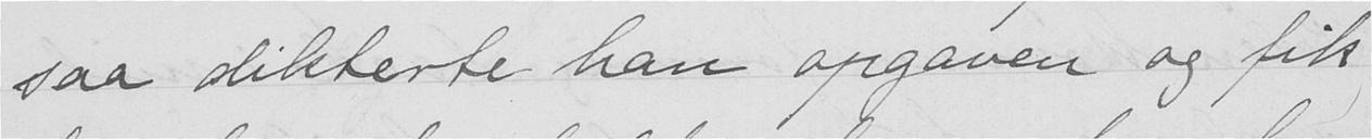saa dikterte han opgaven og fik
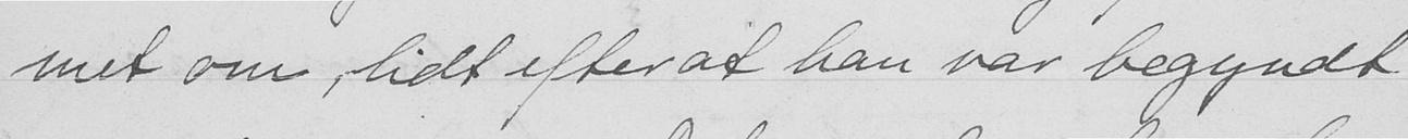met om, lidt efter at han var begyndt
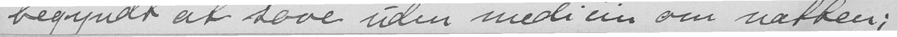begyndt at sove uden medicin om natten;
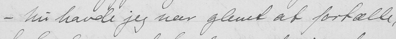- Nu havde jeg nær glemt at fortælle,
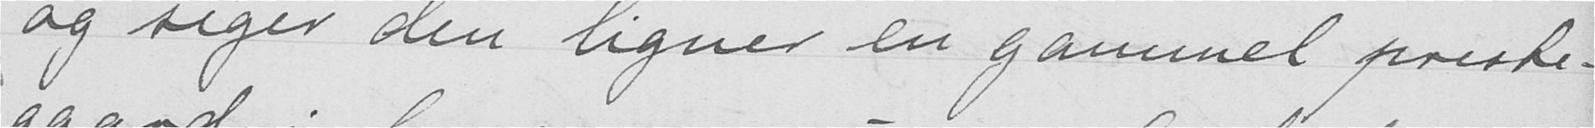og siger den ligner en gammel preste-
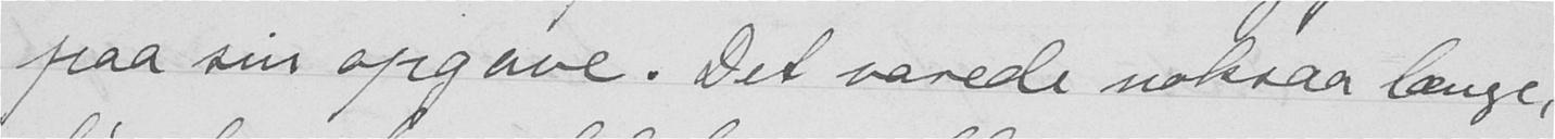paa sin opgave. Det varede noksaa længe,
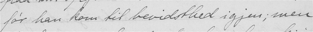før han kom til bevidsthed igjen; men
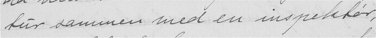tur sammen med en inspektør,
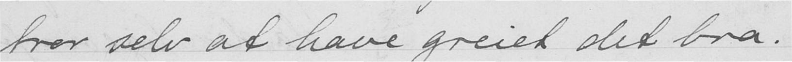tror selv at have greiet det bra.
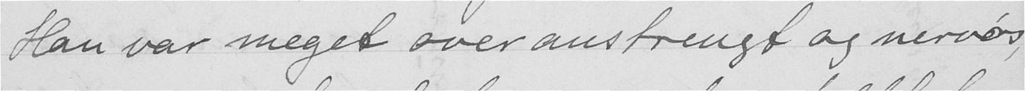Han var meget overanstrengt og nervøs,
